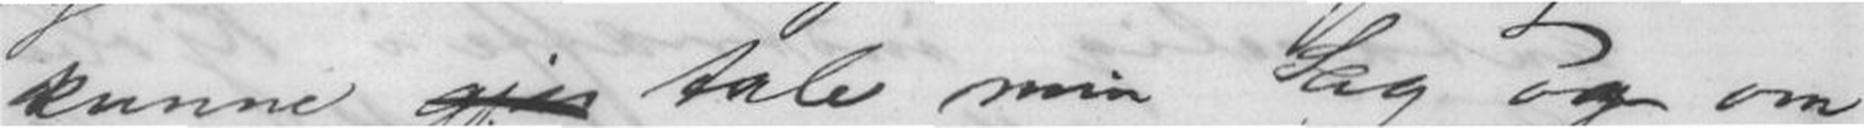kunne gjør tale min Sag og om
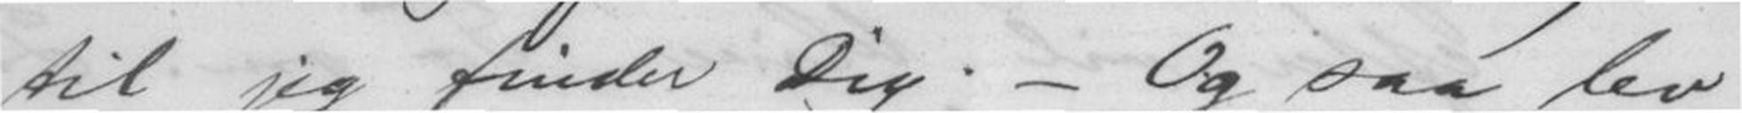til jeg finder Dig. - Og saa lev
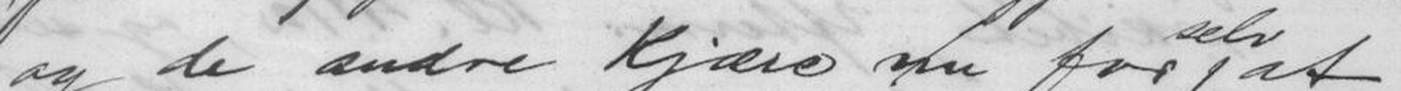og de andre Kjære nu for at
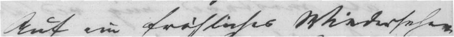Auf im fröhliche Wiedersehen
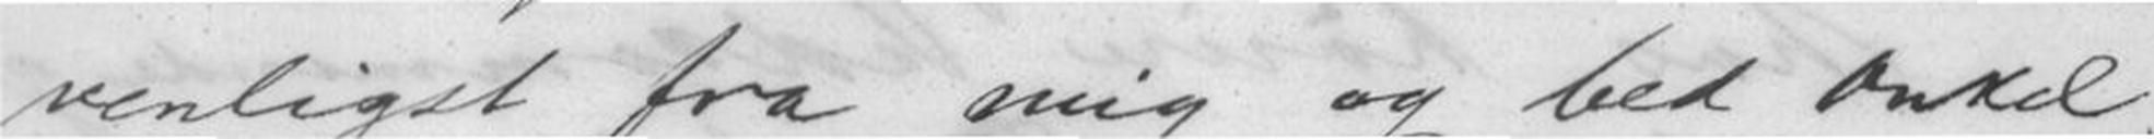venligst fra mig og bed Onkel,
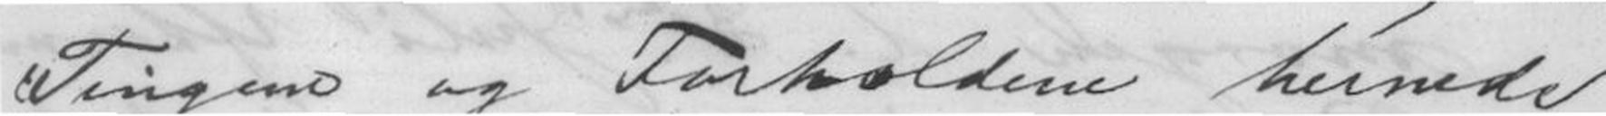Tingene og Forholdene hernede
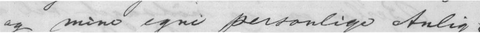og mine egne personlige Anlig-
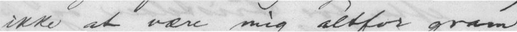ikke at være mig altfor gram,
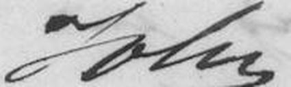John
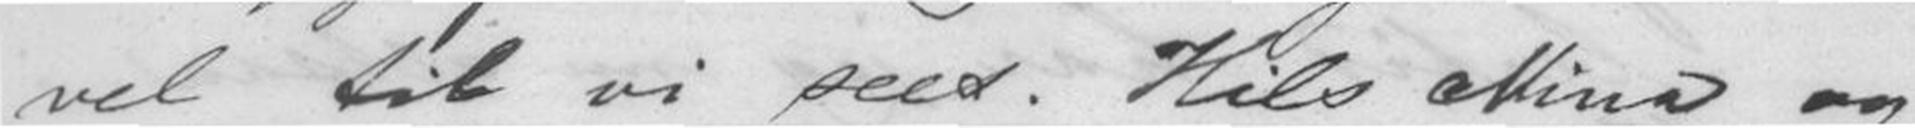vel til vi sees. Hils Nina og
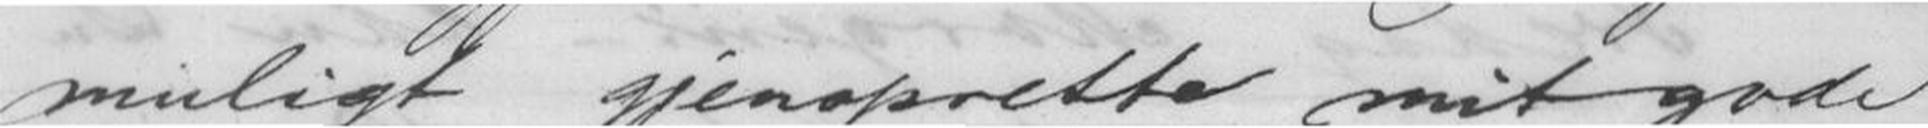mulig gjenoprette mit gode
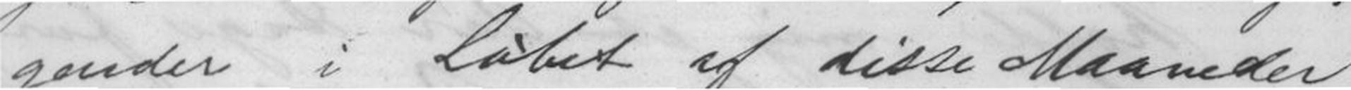gender i Løbet af disse Maaneder
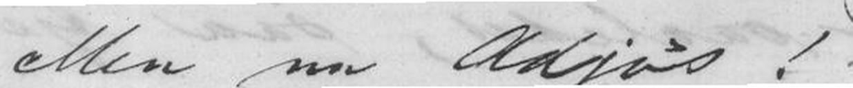Men nu Adjøs!
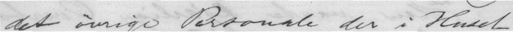det øvrige Personale der i Huset
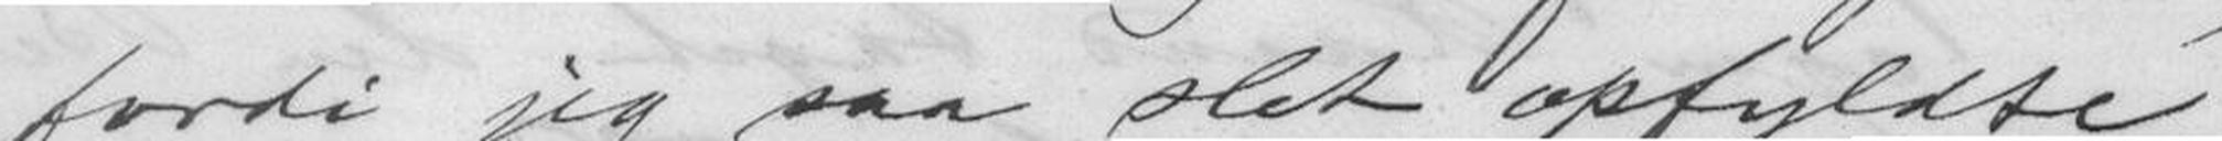fordi jeg saa slet opfyldte
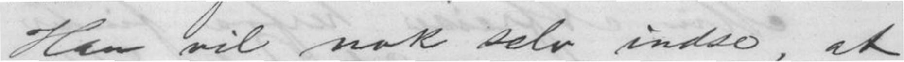Han vil nok selv indse, at
